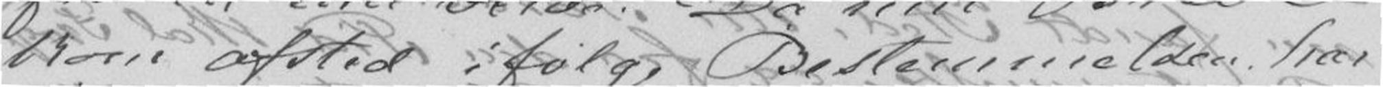kom afsted ifølge Bestemmelsen har
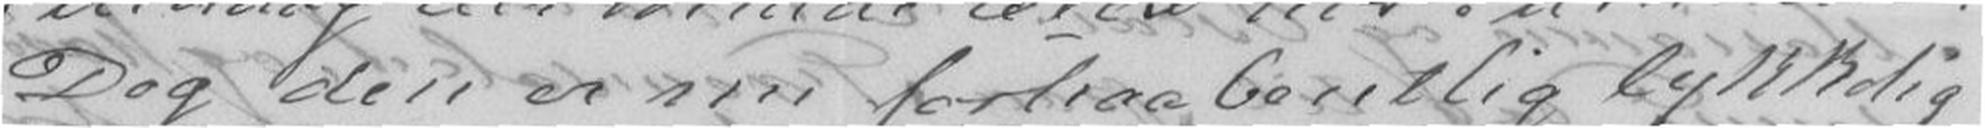Dog den er nu forhaabentlig lykkelig
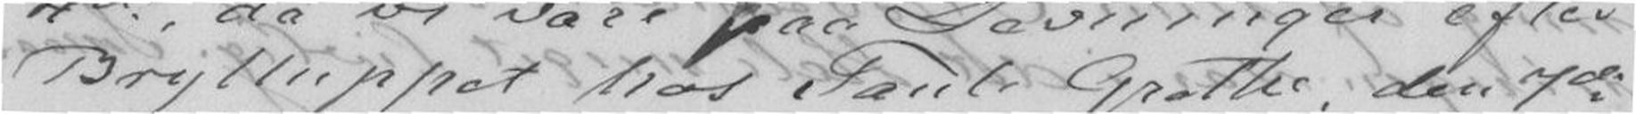Brylluppet hos Tante Grethe, den 7de
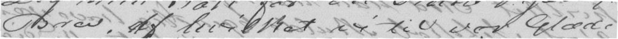Brev, af hvilket vi til vor Glæde
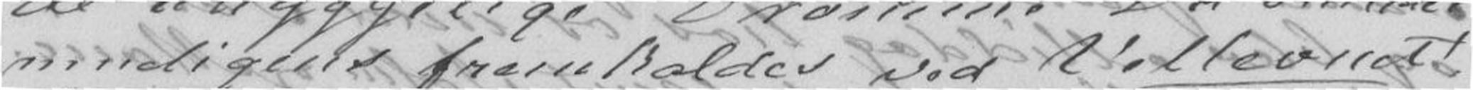mueligens fremholdes ved Vellevud!
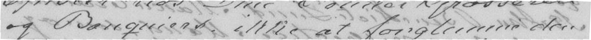og Banquiers ikke at forglemme dens
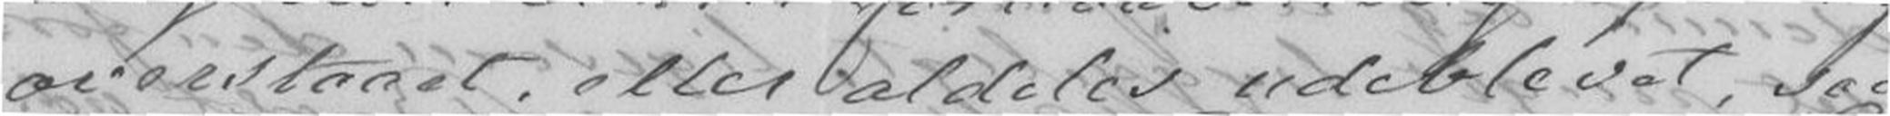overstaaet, eller aldeles udeblevet, saa
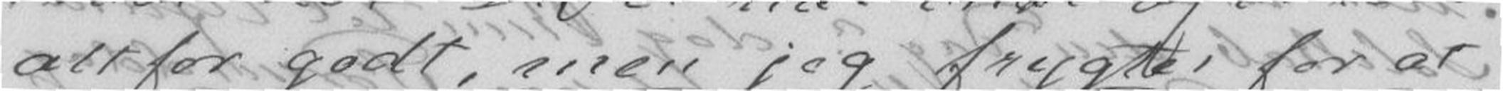alt for godt, men jeg frygter for at
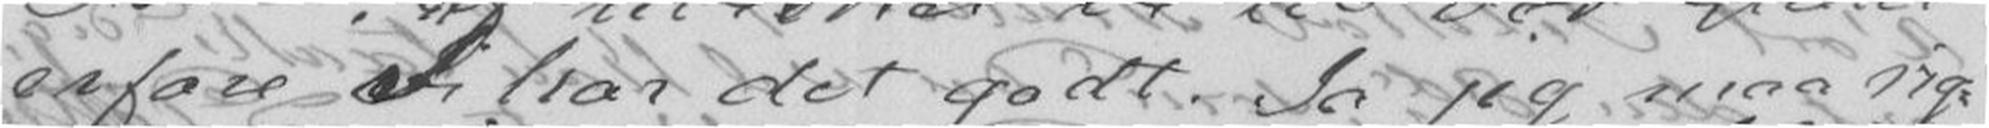erfare vi har det godt. Ja jeg maa rig-
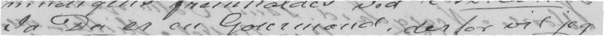Ja Du er en Gourmand, derfor vil jeg
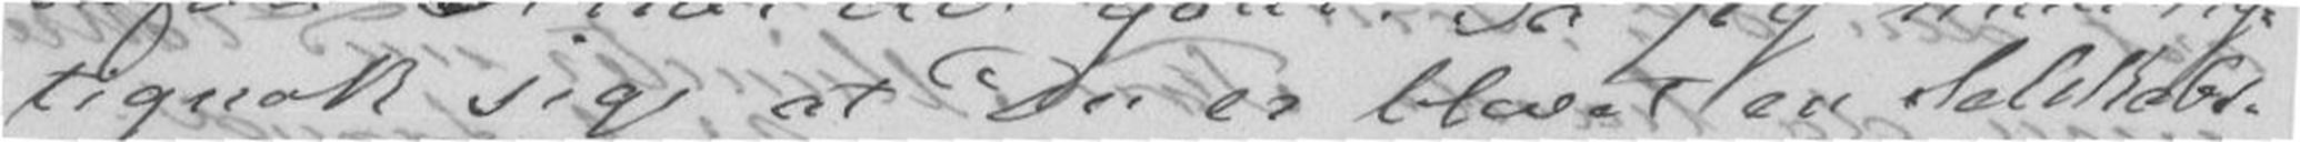tignok sige at Du er blevet en Selskabs-
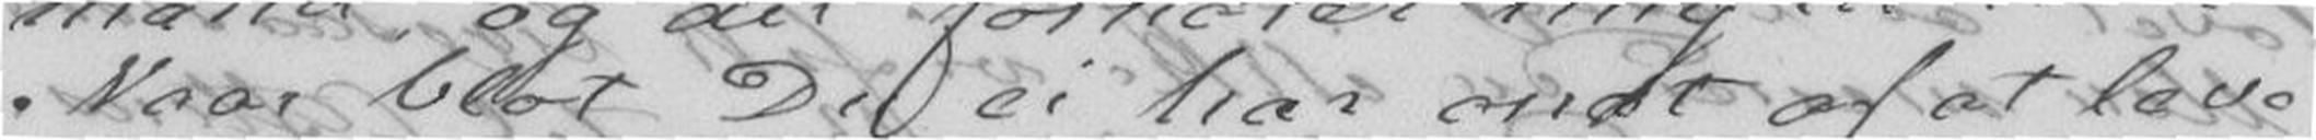Naar blot Du ei har ondt af at leve
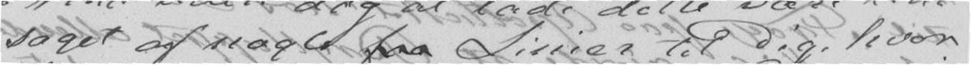saget af nogle faa Linier til Dig hvor
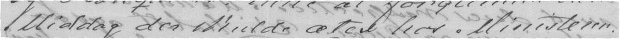Middag der skulde ætes hos Ministeren.
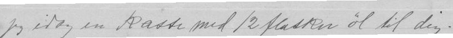jeg idag en kasse med 12 flasker öl til dig.
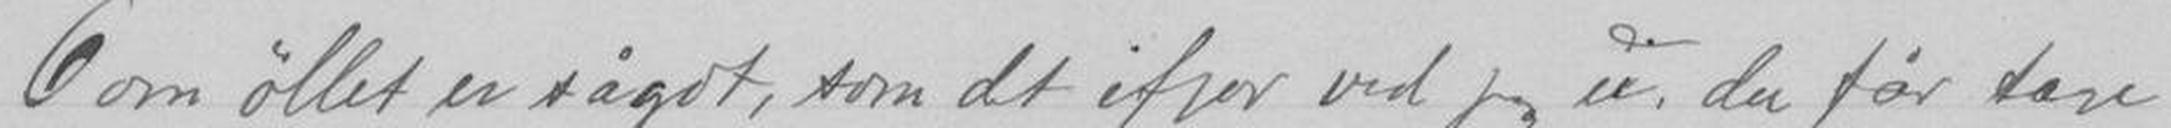Om öllet er sågot, som det ifjor ved jeg ei, du får tave
